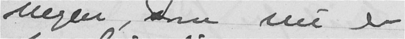ingen, som nu er
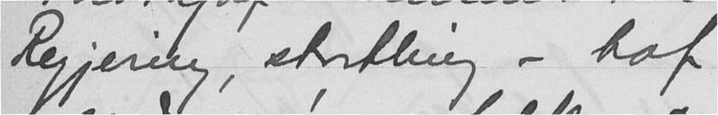Regjering, storthing - hof
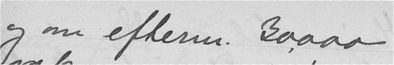og om efterm. 30,000
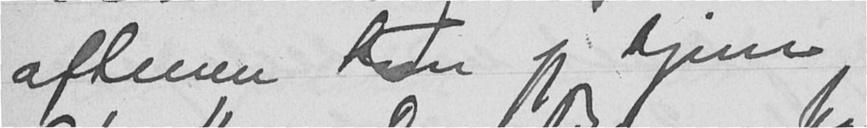aftenen kom jeg hjem
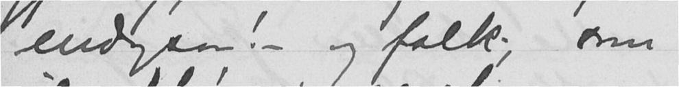endogen! - og folk som
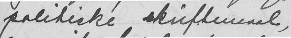politiske skriftemaal,
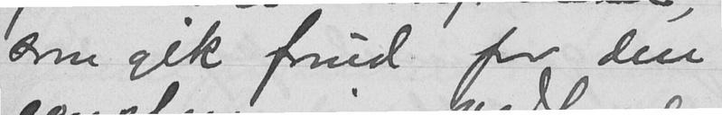som gik forud for den
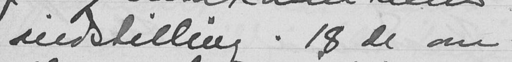indstilling. 18 de om
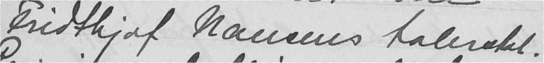Fridtjof Nansens talerstol.
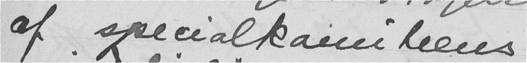af specialkomiteens
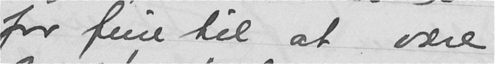for fine til at være
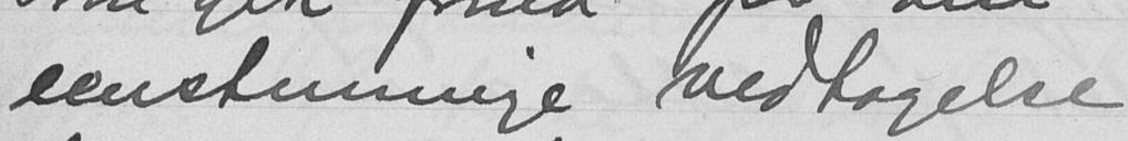eenstemmige vedtagelse
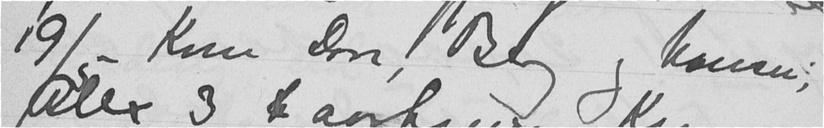19/5 Kom Don, Berg og Nansen;
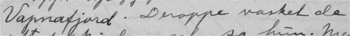Vapnafjord. Deroppe vasket de
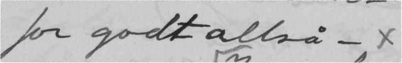for godt altså - x
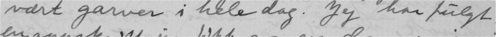vært garver i hele dag. Jeg har fulgt
